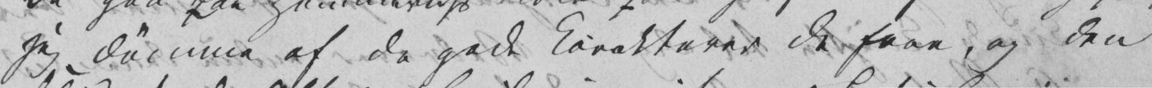jeg dømme af de gode Carakterer de faae, og den
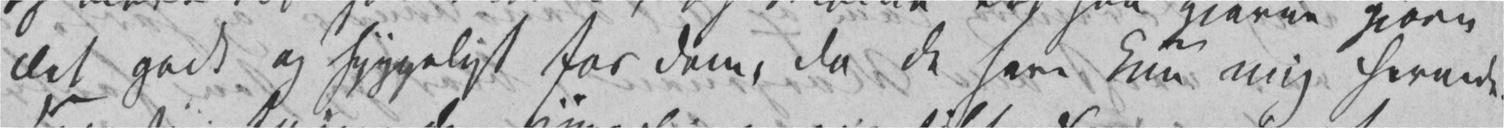det godt og hyggeligt for dem, da de have kun mig hernede.
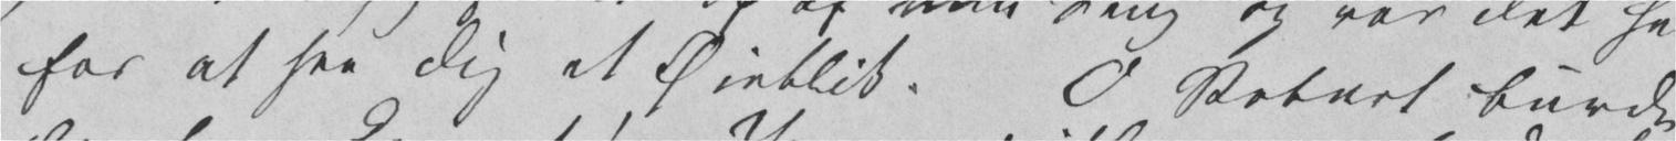for at see Dig et Øieblik. O Robert burde
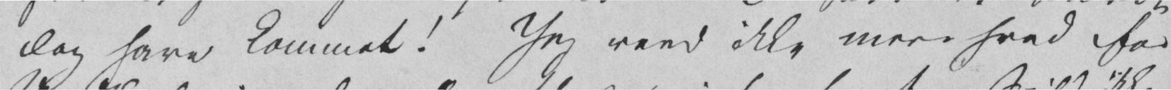dog have kommet! Jeg veed ikke mere hvad for
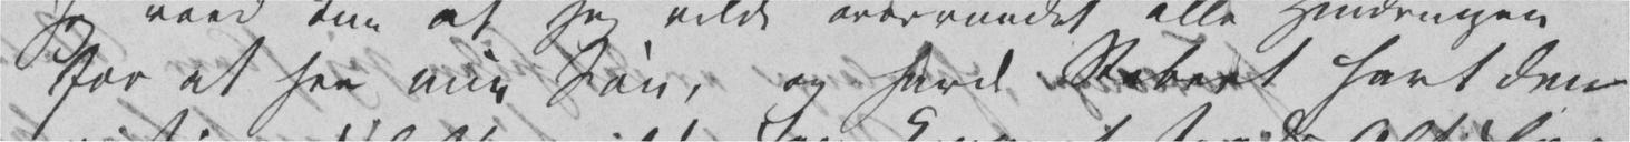for at see min Søn, og havde Robert havt den
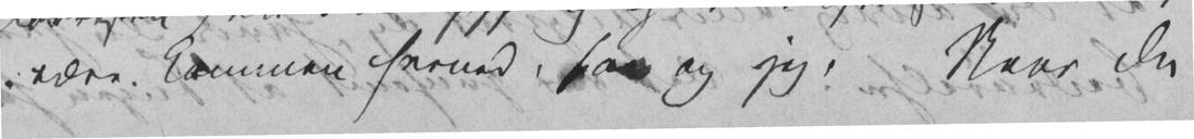være kommen herned, saa og jeg. Naar Du
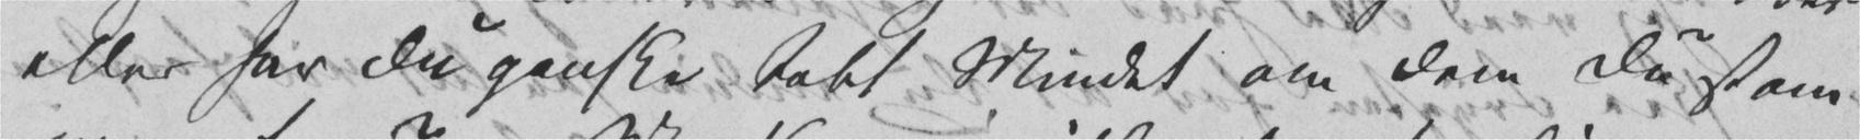eller har Du ganske tabt Mindet om dem Du stam-
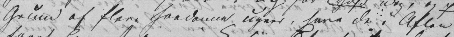Grund af flere saadanne ugeer, have de i Aften
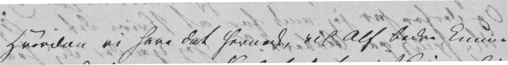Hvordan vi have det hernede, vil Alf bedre kunne
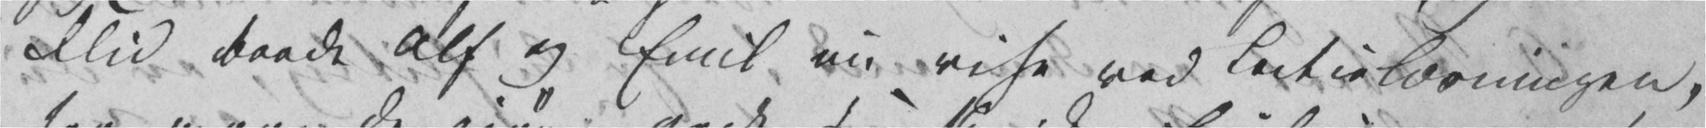Flid baade Alf og Emil nu vise ved Lectielæsningen,
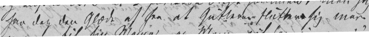har dog den Glæde at see at Gutterne slutter sig mere
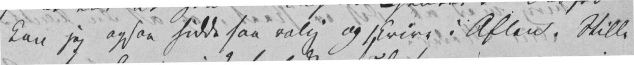kan jeg ogsaa sidde saa rolig og skrive i Aften. Stille
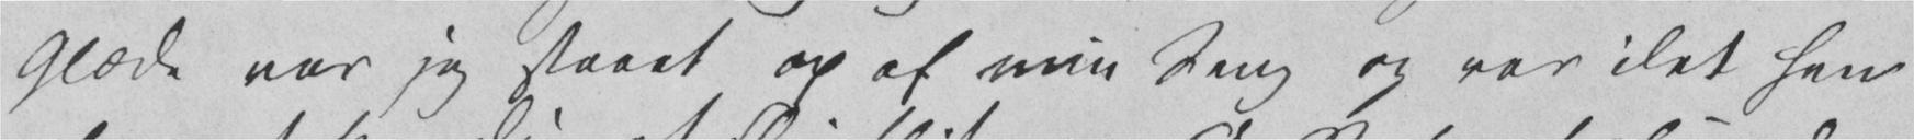Glæde var jeg staaet op af min Seng og var ilet hen
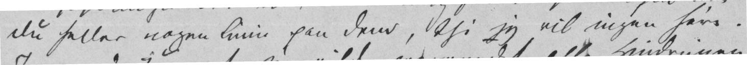Du heller nogen Linie paa dem, thi jeg vil ingen høre.
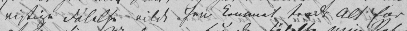rigtige Følelse vilde han kommet trods Alt for
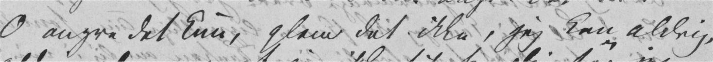O angre det kun, glem det ikke, jeg kan aldrig,
